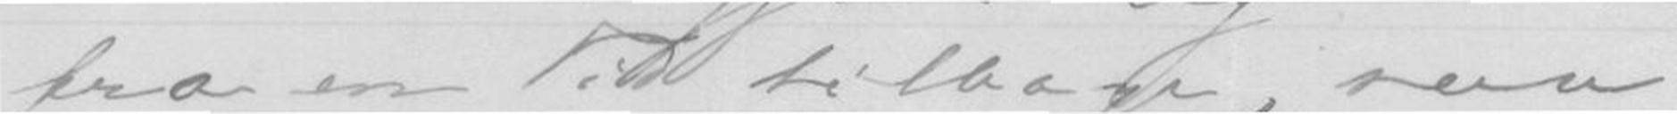fra en Tid tilbage, saa
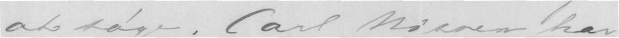at søge. Carl Nissen har
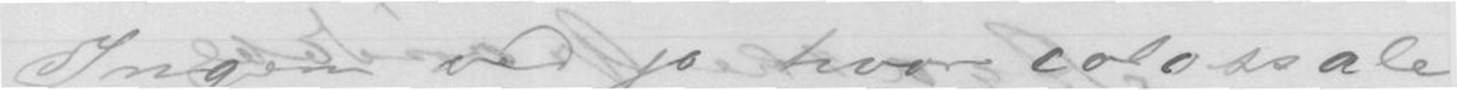Ingen ved jo hvor colossale
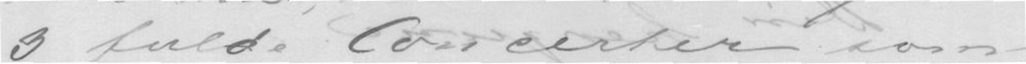3 fulde Concerter som-
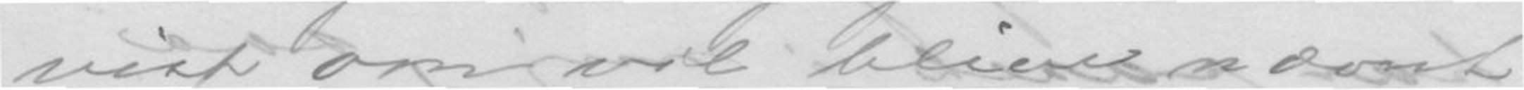vist om vil blive nævnt
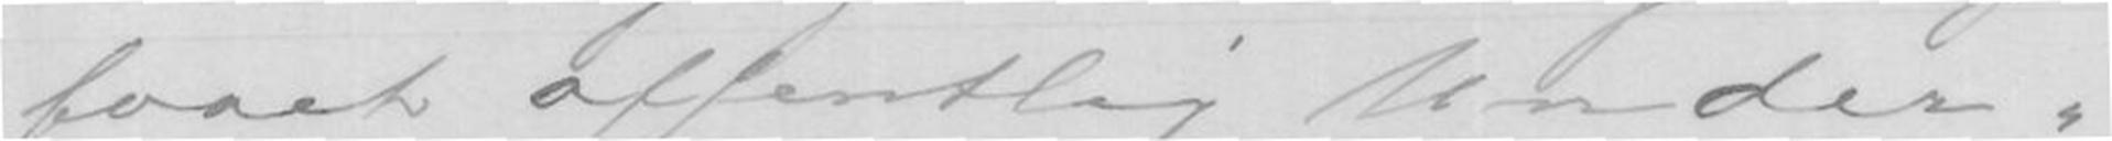faaet offentlig Under-
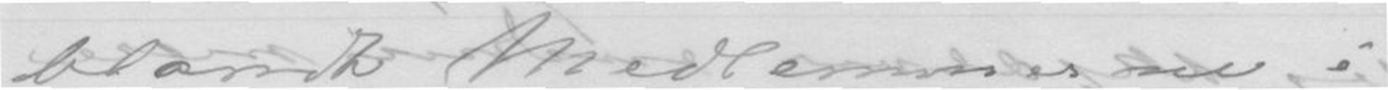blandt Medlemmerne i
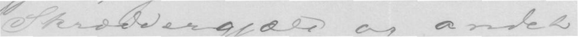Skræddergjæld og andet
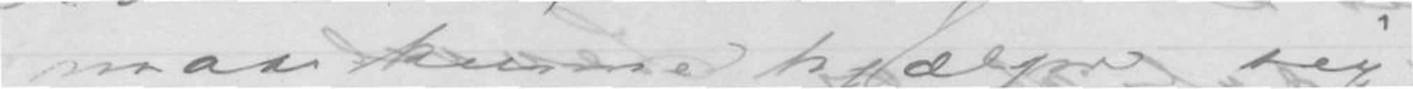maa kunne hjælpe sig
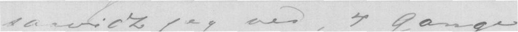saavidt jeg ved, 4 Gange
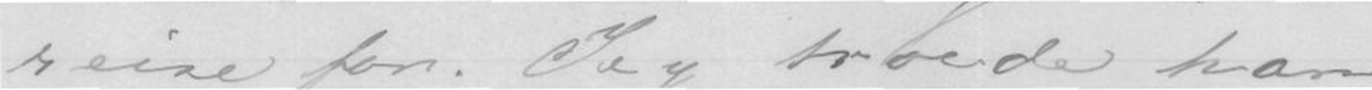reise for. Jeg troede han
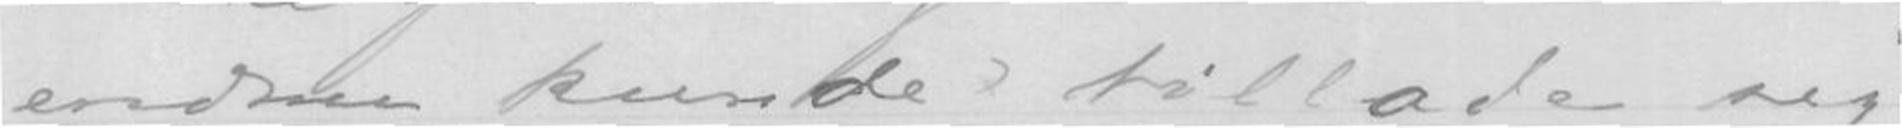endnu kunde tillade sig
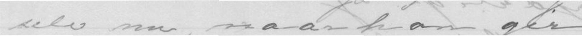selv nu, naar han gir
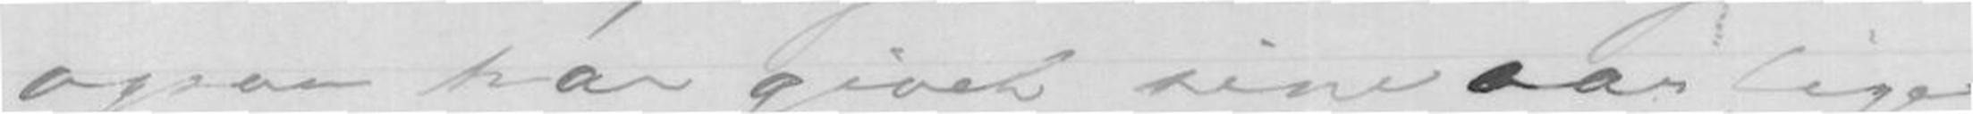ogsaa har givet sine aarlige
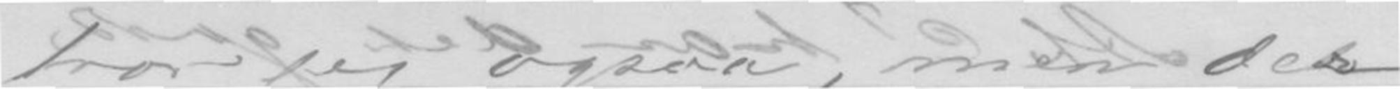tror jeg ogsaa, men det
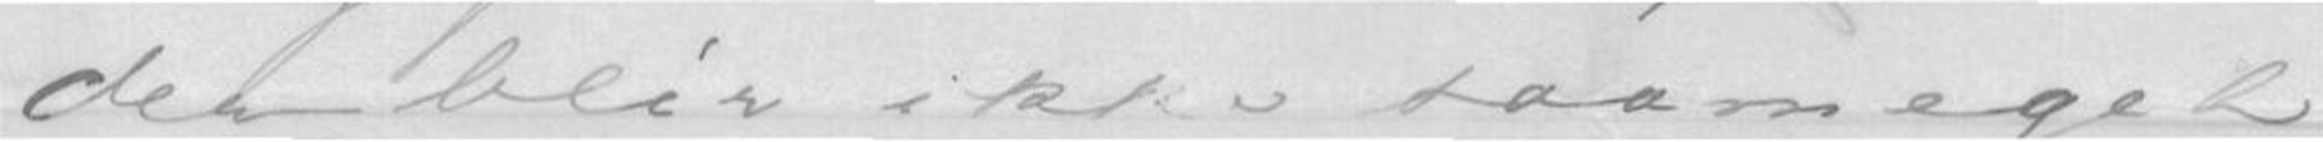der blir ikke saameget
